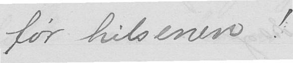for hilsenen!
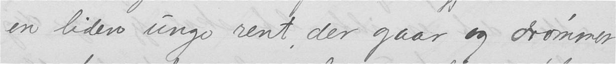en liden unge rent, der gaar og drømmer
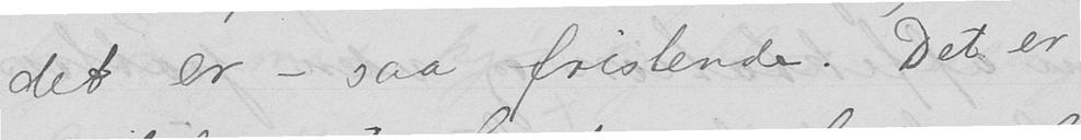det er - saa fristende. Det er
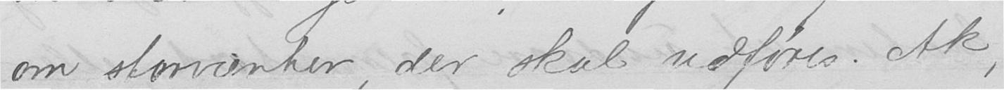om storværker, der skal udføres. Ak,
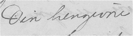Din hengivne
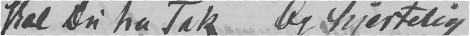skal Du ha Tak Og hjertelig
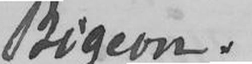Bigeon.
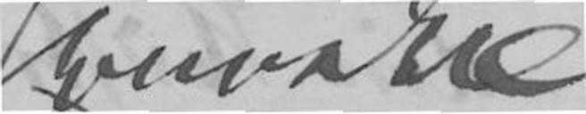Jonas Lie.
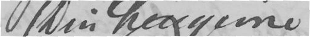Din hengivne
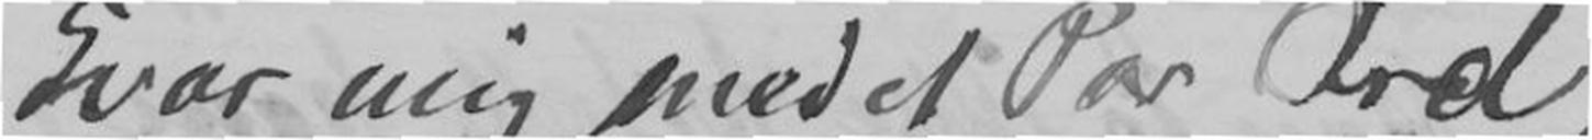Svar mig med et Par Ord,
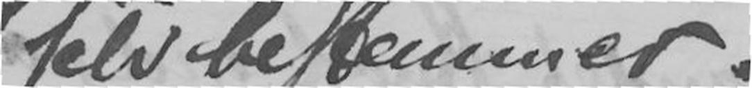I selv bestemmer.
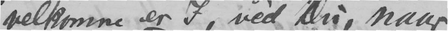velkomne er I, ved Du, naar
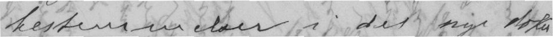bestemmelser i, det nye doku-
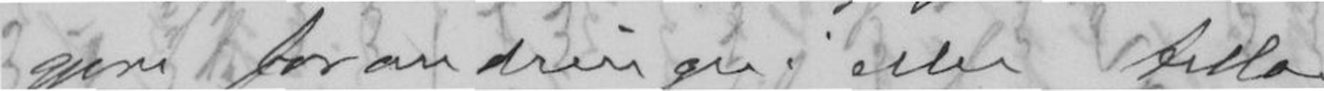gjøre forandringer eller tillæg
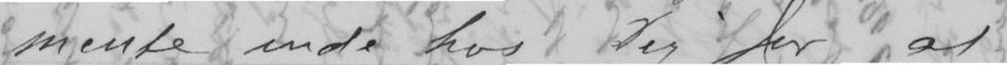mentet inde hos Dig før at
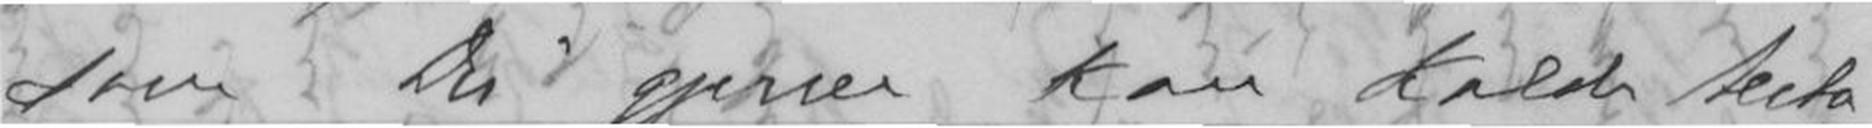som Du gjerne kan kalde testa-
